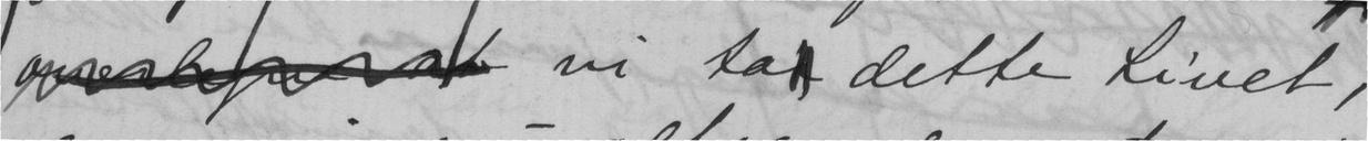vi ta dette Livet,
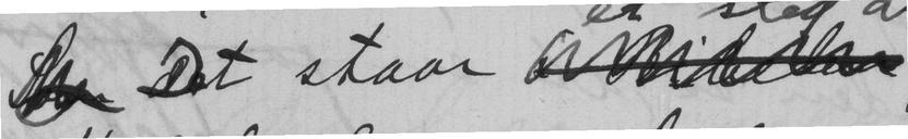Det staar
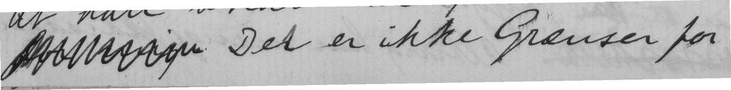Det er ikke Grænser for
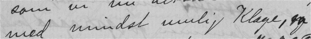med mindst mulig Klage,
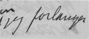jeg forlanger
